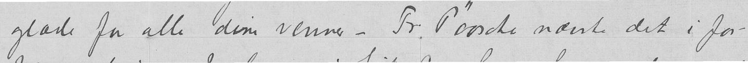glæde for alle dine venner – Fr. Paasche nævte det i for-
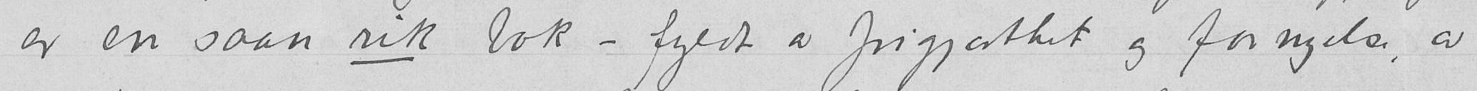er en saan rik bok – fyldt av frigjorthet og fornyelse, av
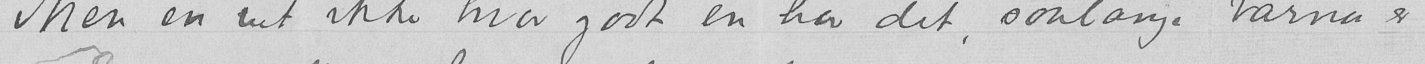Men en vet ikke hvor godt en har det, saalænge barna er
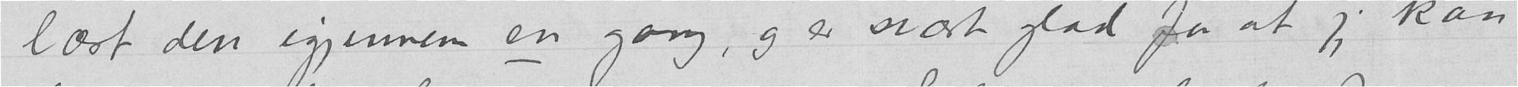læst den igjennem en gang, og er svært glad for at jeg kan
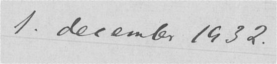1. december 1932.
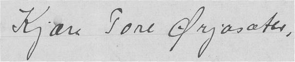Kjære Tore Ørjasæter,
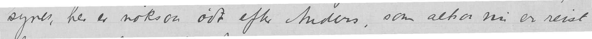synes, her er noksaa ødt efter Anders, som altsaa nu er reist
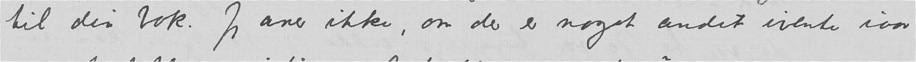til din bok. Jeg aner ikke, om der er noget andet ivente iaar
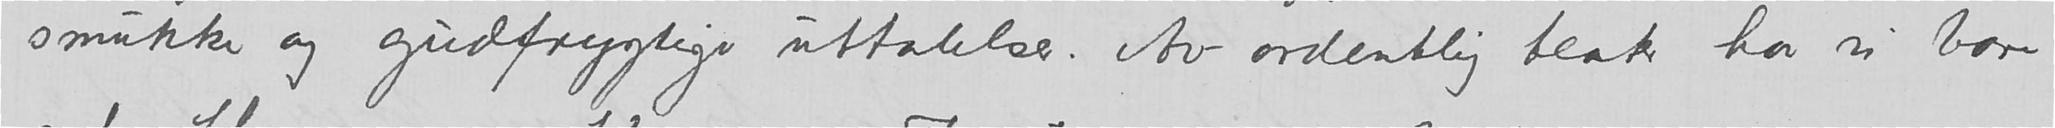smukke og gudfrygtige uttalelser. Av ordentlig teater har vi bare
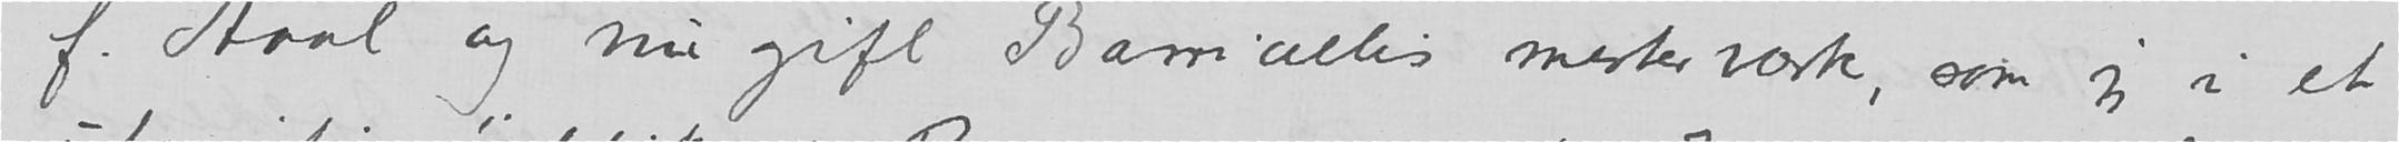f. Aaal og nu gift Barri celli mesterværk, som jeg i et
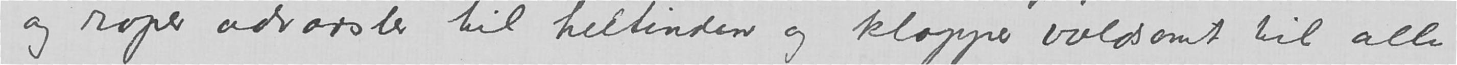og roper advarsler til heltinden og klapper voldsomt til alle
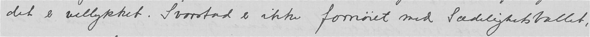det er vellykket. Svarstad er ikke fornøiet med Sædelighetsballet,
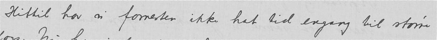Hittil har vi forresten ikke hat tid engang til større
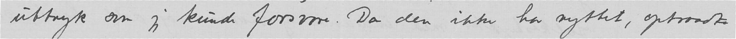uttryk som jeg kunde forsvore. Da den ikke har n	yttet, optraadte
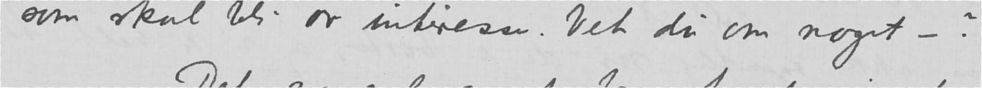som skal bli av interesse. Vet du om noget –?
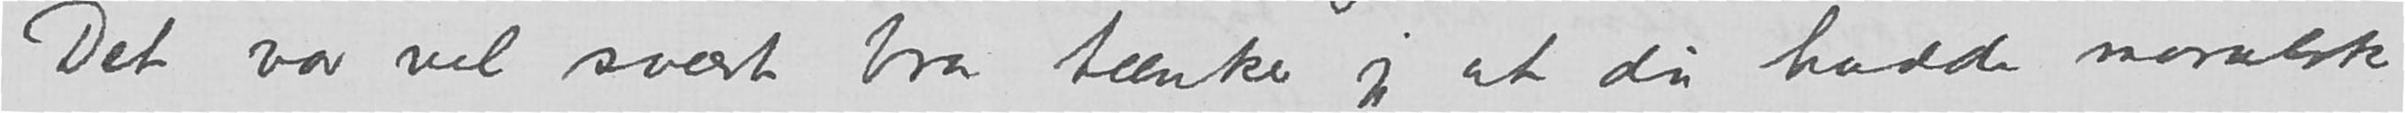Det var vel svært bra tænker jeg at du hadde moralsk
